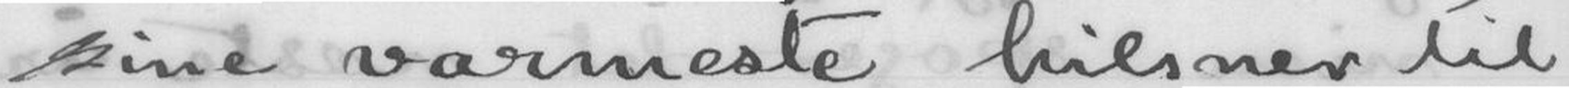sine varmeste hilsner til
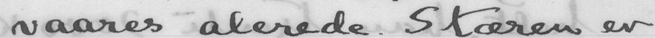vaares alerede. Stæren er
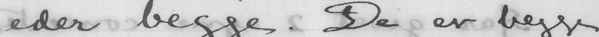eder begge. De er begge
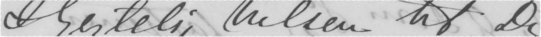Hjertelig hilsen til Dig
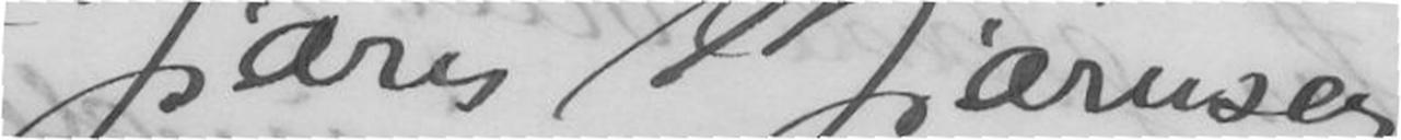Bjørn Bjørnson
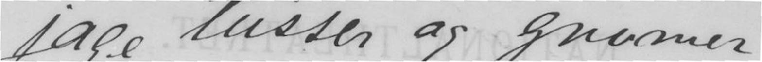jage tusser og gnomer
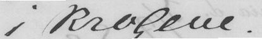i krogene.
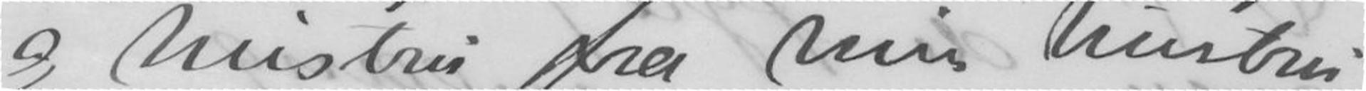og hustru fra min hustru
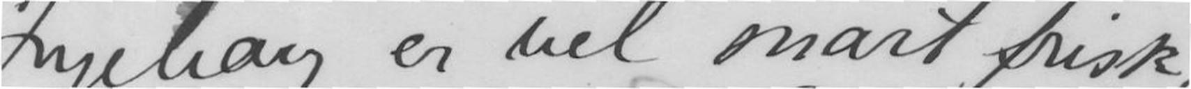Ingeborg er vel snart frisk,
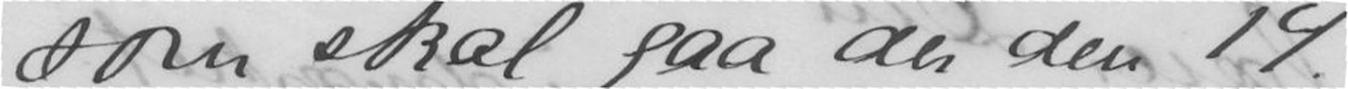som skal gaa der den 14.
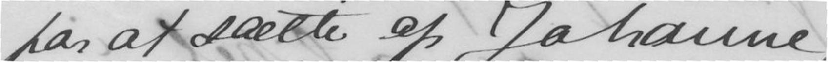for at sætte op Johanne,
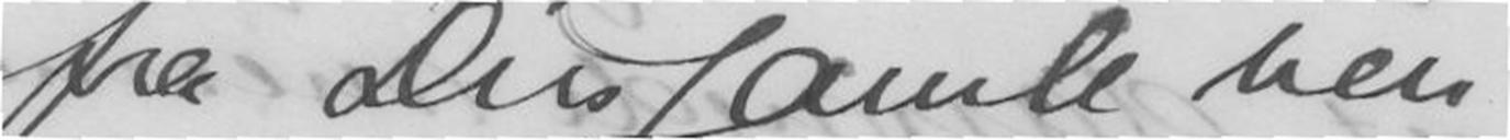og fra Din gamle ven
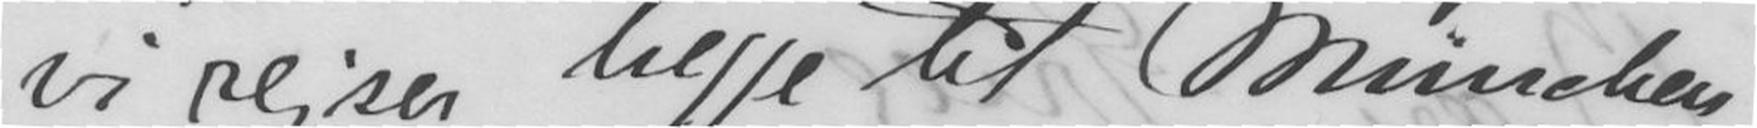vi rejser begge til Munchen
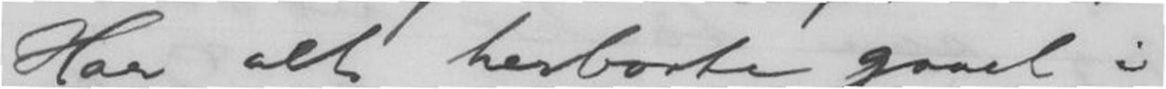Har alt herborte gaaet i
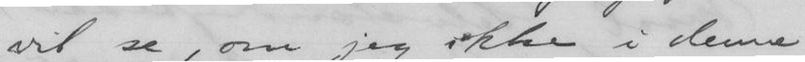vil se, om jeg ikke i denne
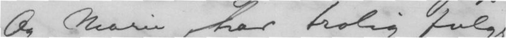Og Marie har trolig fulgt
